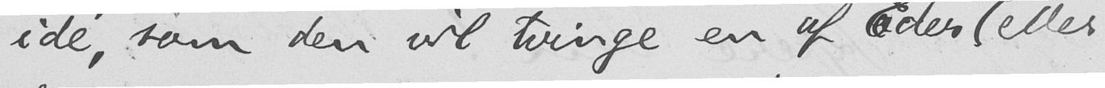idé, som den vil tvinge en af Eder (eller
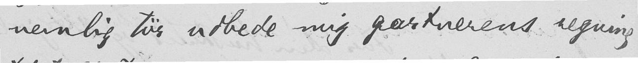nemlig tør udbede mig gartnerens regning
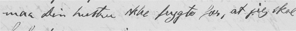maa Din hustru ikke frygte for, at jeg skal
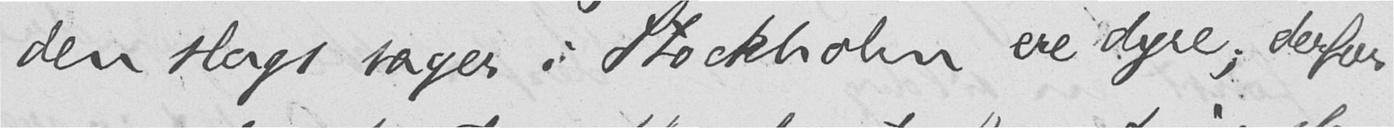den slags sager i Stockholm ere dyre; derfor
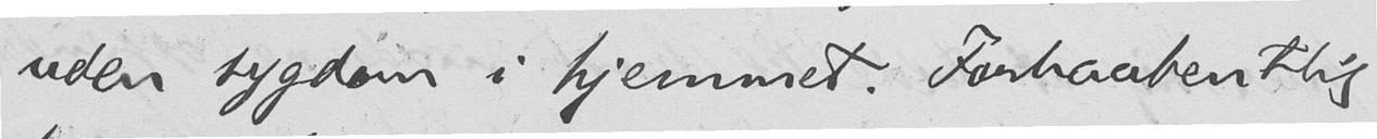uden sygdom i hjemmet. Forhaabentlig
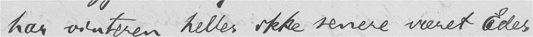har vinteren heller ikke senere været Eder
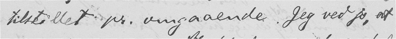tilstillet pr. omgaaende. Jeg ved jo, at
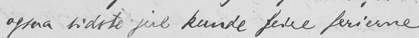ogsaa sidste jul kunde feire ferierne
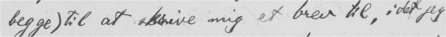begge) til at skrive mig et brev til, idet jeg
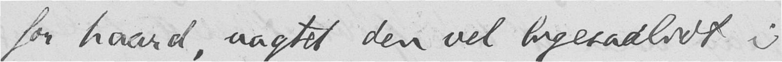for haard, uagtet den vel ligesaavidt i
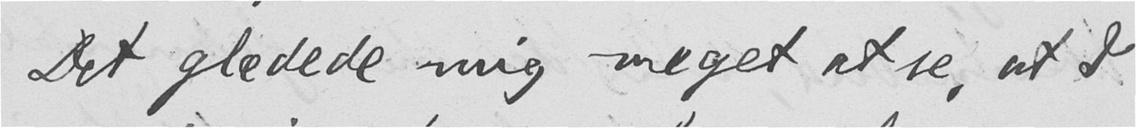Det gledede mig meget at se, at I
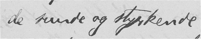de sunde og styrkende.
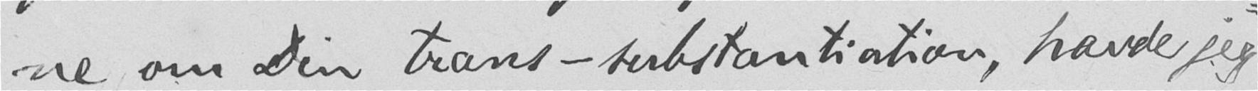ne om Din trans–substantiation, havde jeg
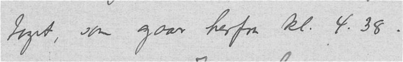toget, som gaar herfra kl. 4.38.
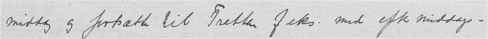middag og fortsætte til Tretten f eks. med eftermiddags-
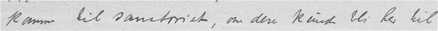kommer til sanatoriet, om dere kunde bli her til
